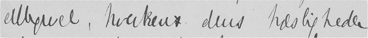alligevel, hverken dens hæsligheder
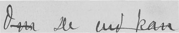Om De end kan
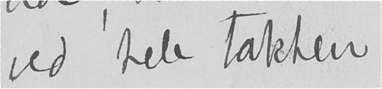ved hele takken
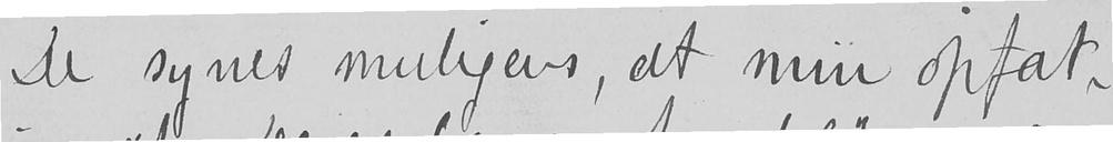De synes muligens at min opfat-
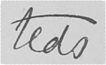teds
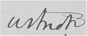vistnok
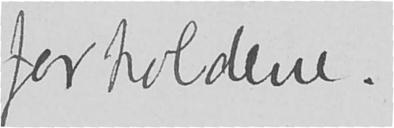forholdene.
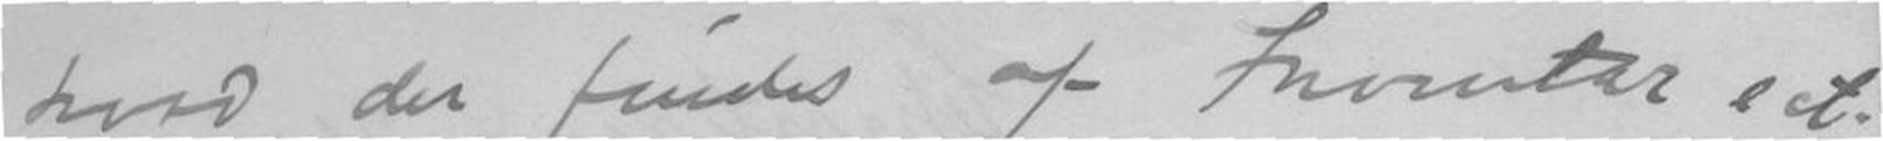hvad der findes af Inventar e ct.
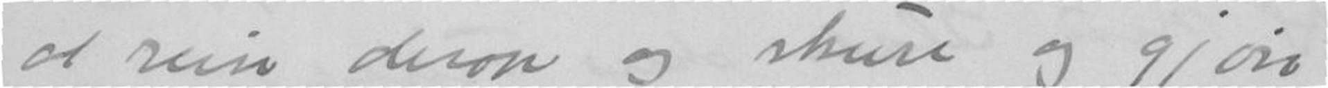at reise derop og skure og gjøre
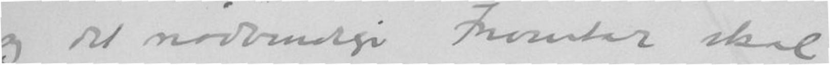og det nødvendige Inventar skal
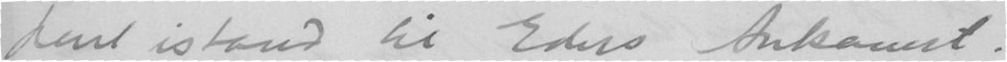den istand til Eders Ankomst.
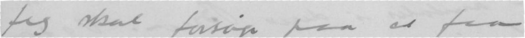Jeg skal forsøge paa at faa
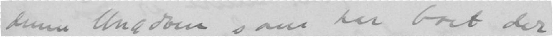denne Ungdom, som har boet der
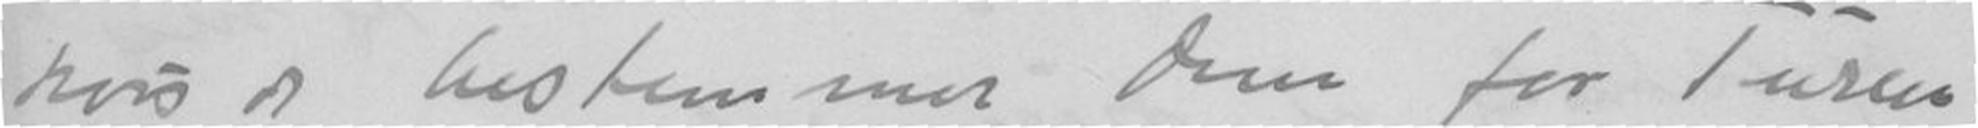hvis de bestemmer Dem for Turen,
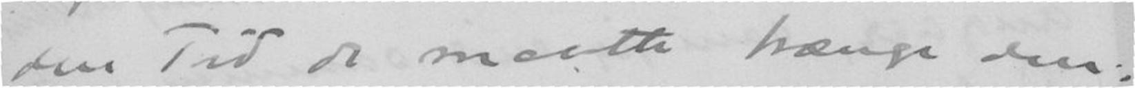den Tid de maatte trænge den.
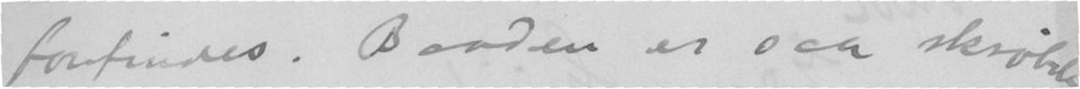forfindes. Baaden er saa skrøbelig
